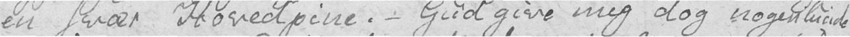en svær Hovedpine. – Gud give mig dog nogenlunde
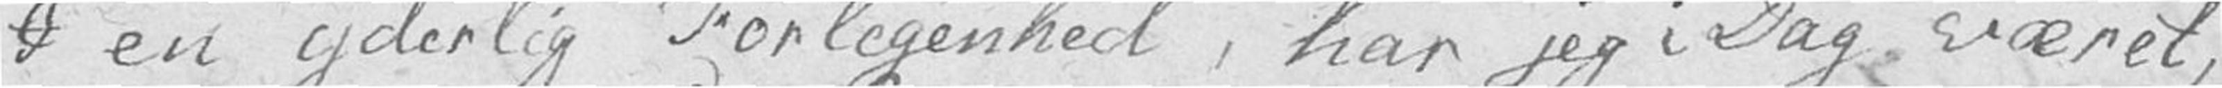I en yderlig Forlegenhed, har jeg i Dag været,
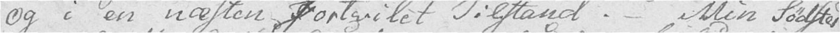og i en næsten fortvilet Tilstand. – Min Sødster
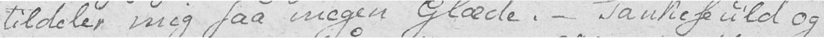tildeler mig saa megen Glæde. – Tankefuld og
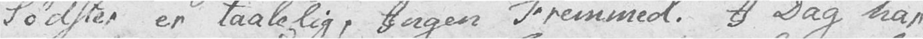Sødster er taalelig, Ingen Fremmed. I Dag har
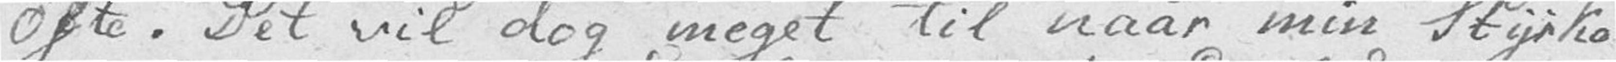ofte. Det vil dog meget til naar min Styrke
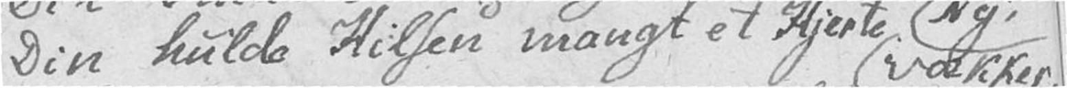Din hulde Hilsen mangt et Hjerte vækker.
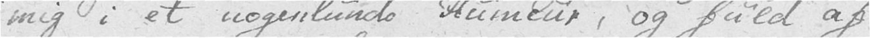mig i et nogenlunde Humeur, og fuld af
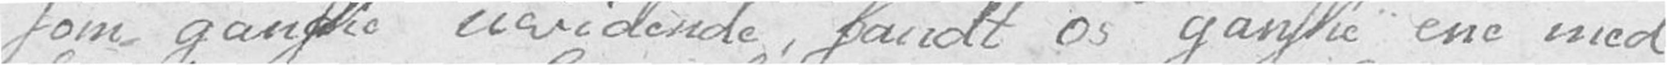som ganske uvidende, fandt os ganske ene med
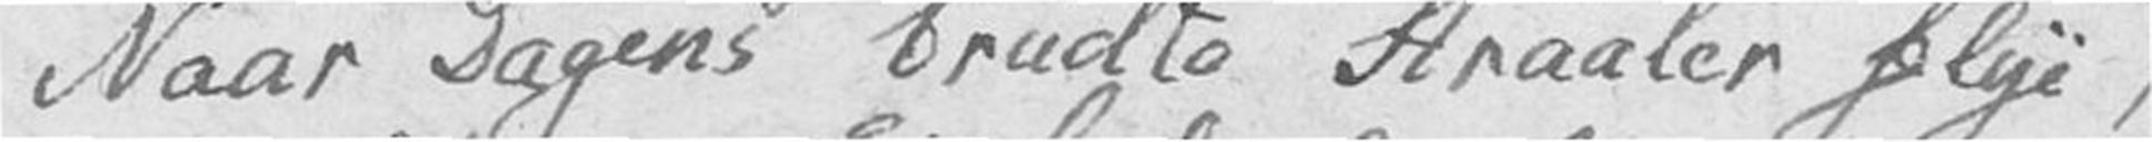Naar Dagens brudte Straaler flye,
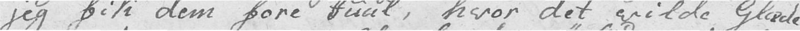jeg fik dem fore Juul, hvor det vilde Glæde
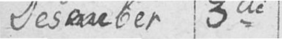Desember 3de
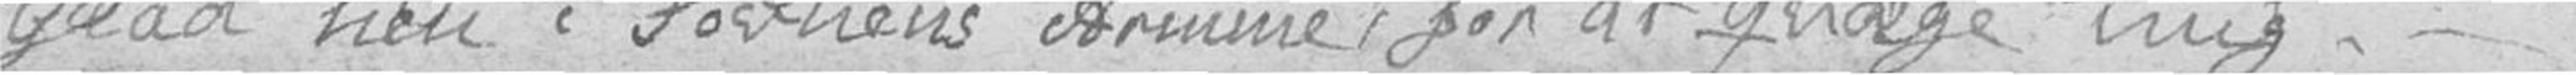Glad hen i Søvnens Armme, for at qvæge mig. –
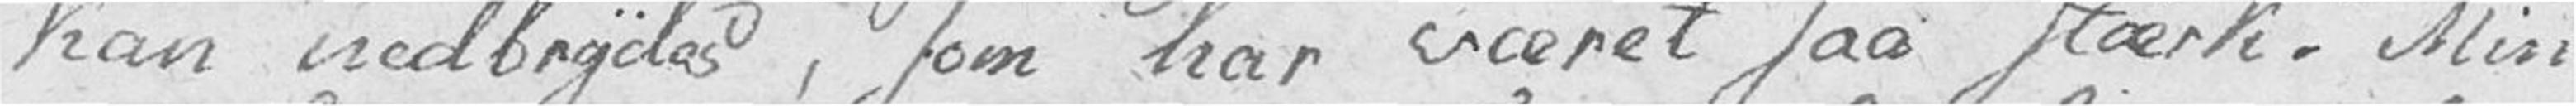kan nedbrydes, som har været saa stærk. Min
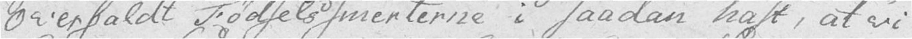overfaldt Fødselssmerterne i saadan hast, at vi
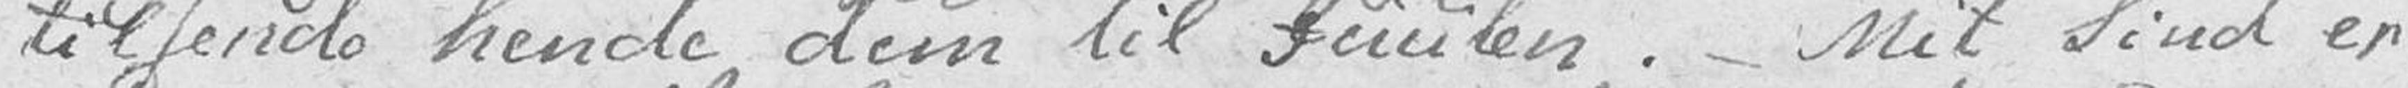tilsende hende dem til Juulen. – Mit Sind er
